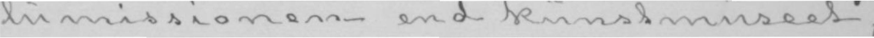lumissionen end kunstmuseet,
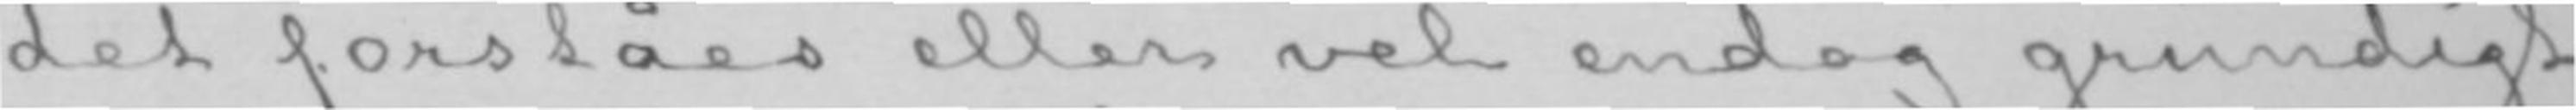det forståes eller vel endog grundigt
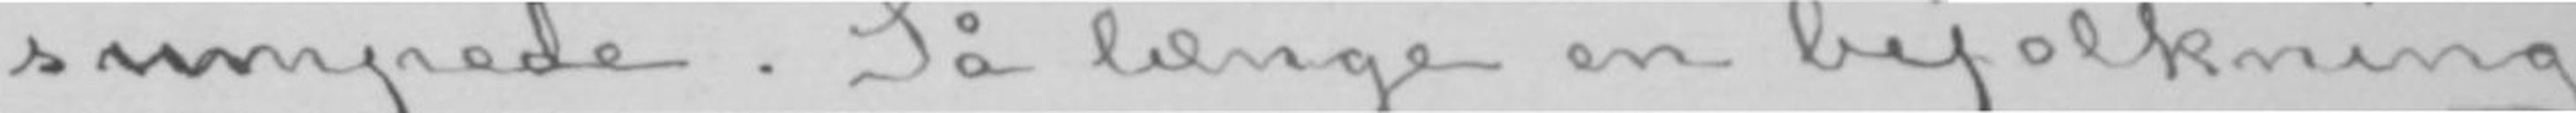sumpede. Så længe en befolkning
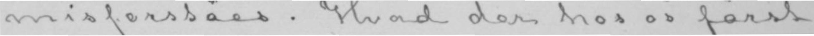misforståes. Hvad der hos os først
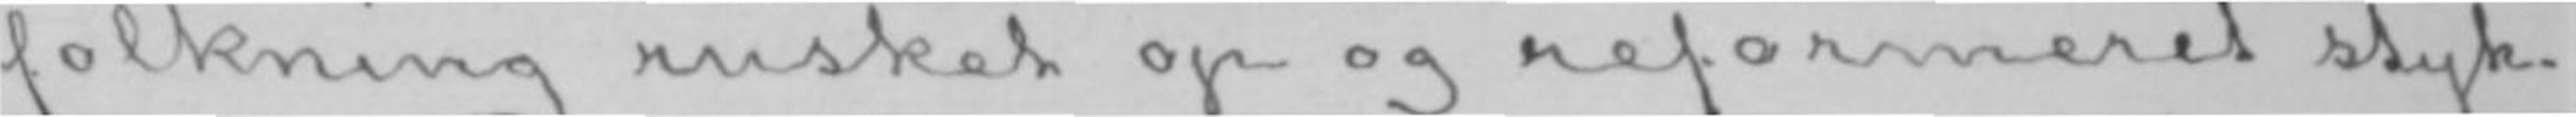folkning rusket op og reformeret styk-
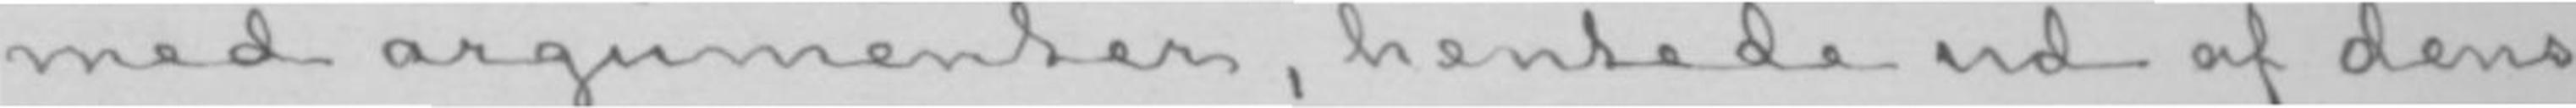med argumenter, hentede ud af dens
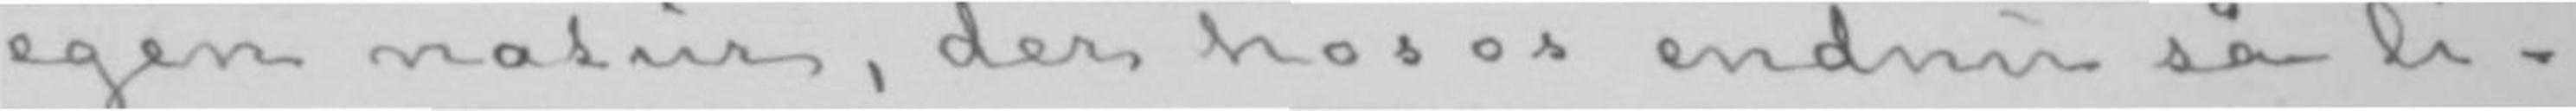egen natur, der hos os endnu så li-
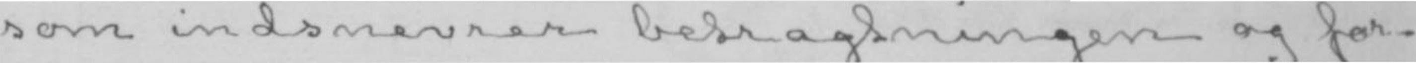som indsnevrer betragtningen og for-
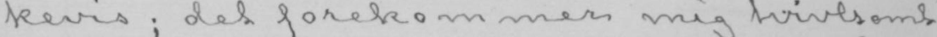kevis; det forekommer mig tvivlsomt
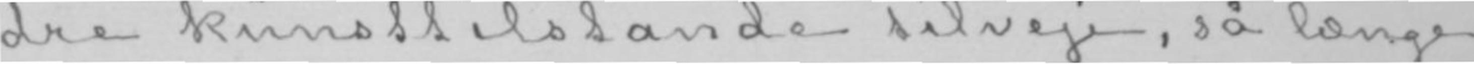dre kunsttilstande tilveje, så længe
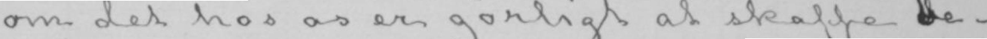om det hos os er gørligt at skaffe be-
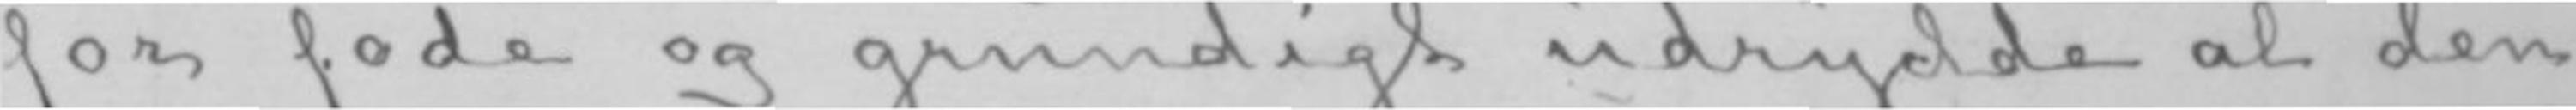for fode og grundigt udrydde al den
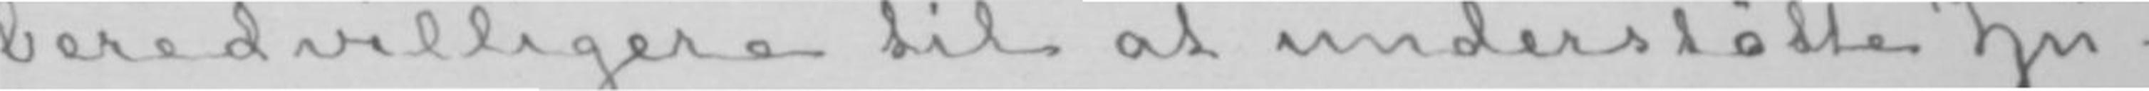beredvilligere til at understøtte Zu-
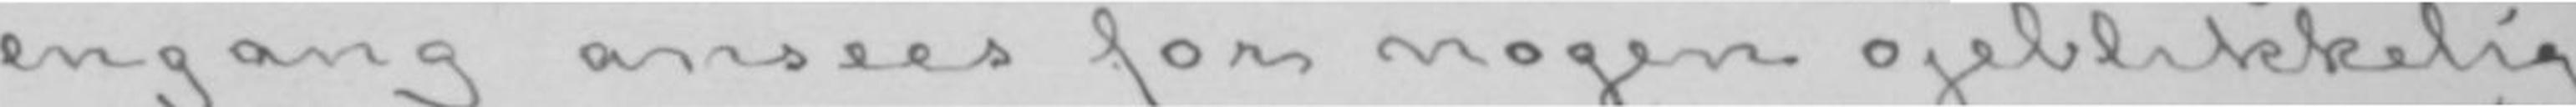engang ansees for nogen øjeblikkelig
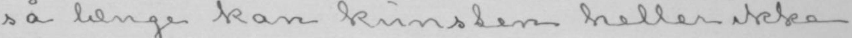så længe kan kunsten heller ikke
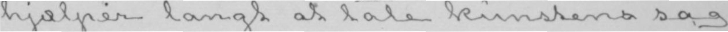hjælper langt at tale kunstens sag
